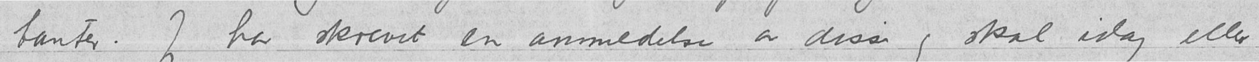tanter. Jeg har skrevet en anmedelse av disse og skal idag eller
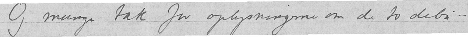Og mange tak for oplysningerne om de to debu –
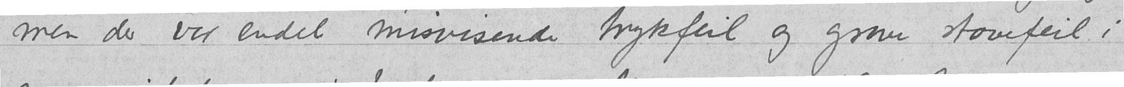men der var endel misvisende trykfeil og grove stavefeil i
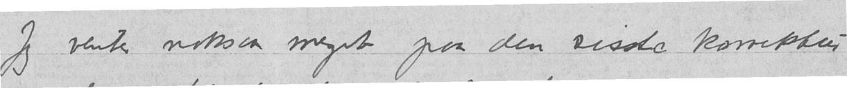Jeg venter noksaa meget paa den sisste korrektur
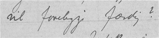vil foreligge færdig?
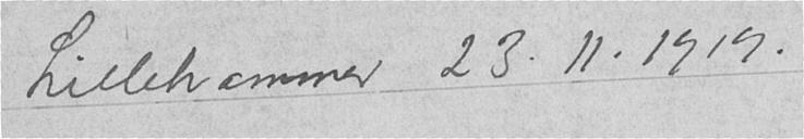Lillehammer 23.11.1919.
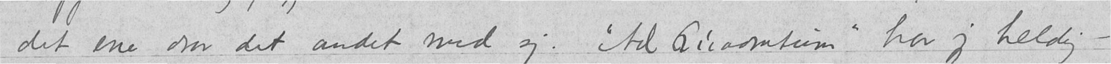det ene drar det andet med sig. "Ad Gicaomtum" har jeg heldig-
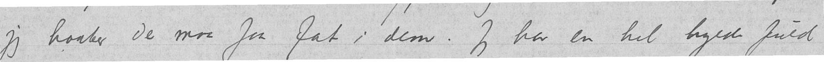jeg haaber De maa faa fat i dem. Jeg har en hel hylde fuld
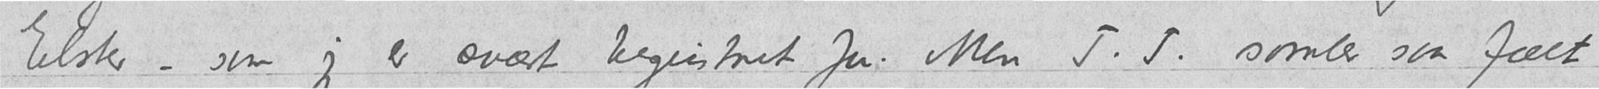Elster – som jeg er svært begeistret for. Men T.T. somler saa fælt
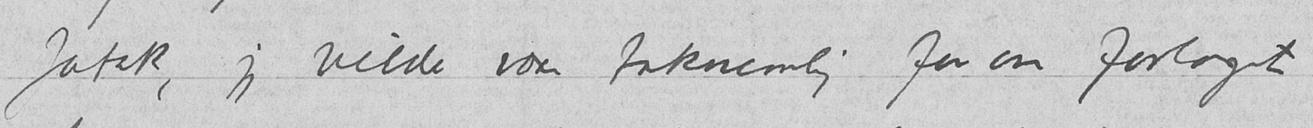Jatak, jeg vilde være taknemlig for om forlaget
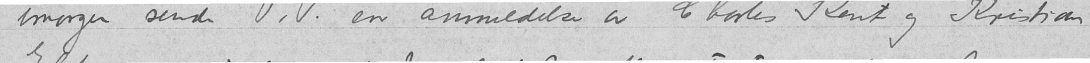imorgen sende T.T. en anmeldelse av Charles Kent og Kristian
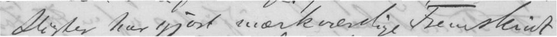Digter har gjort mærkværdige Fremskridt
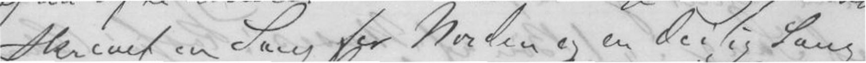Skrevet en Sang for Norden og en deilig Sang
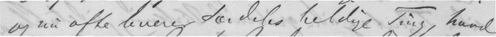og nu ofte leverer særdeles heldige Ting, havde
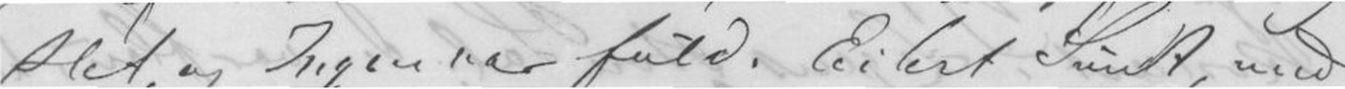slet, og Ingen var fuld. Eilert Sundt, med
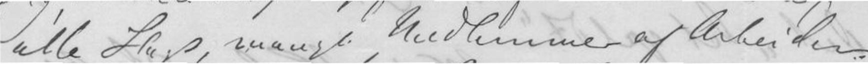alle Slags, mange Medlemmer af Arbeider-
